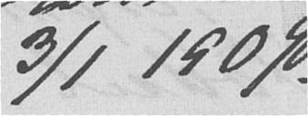3/1 1909.
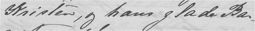Kristen, og hans glade Bar-
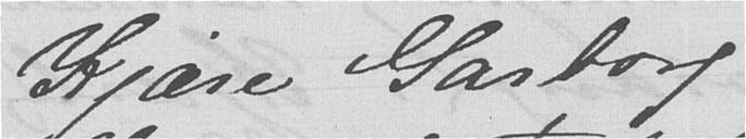Kjære Garborg
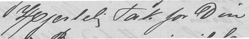Hjertelig Tak for Din
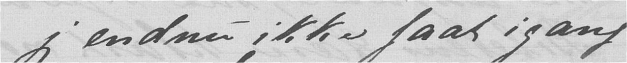jeg endnu ikke faat igang,
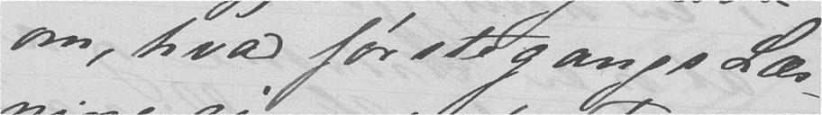om, hvad førstegangs Læs-
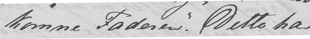komne Faderen. Dette har
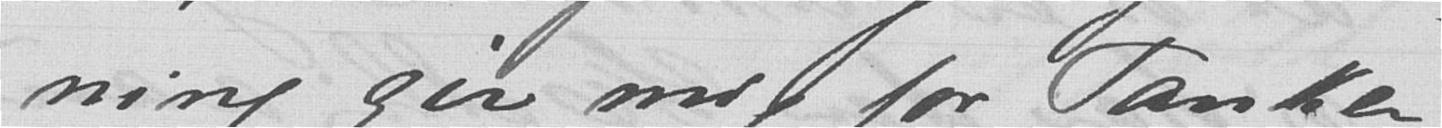ning gir mig for Tanker.
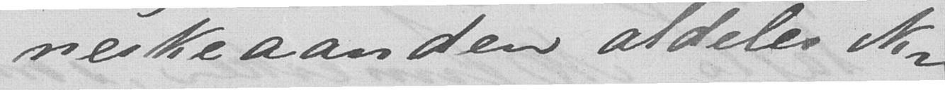neskeaanden aldeles ik-
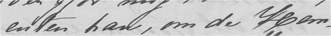enten han, om de Hem-
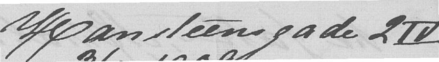Hansteensgade 2 IV
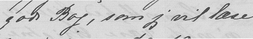gode Bog, som jeg vil læse
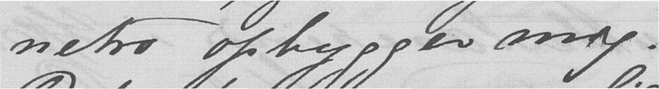netro opbygger mig.
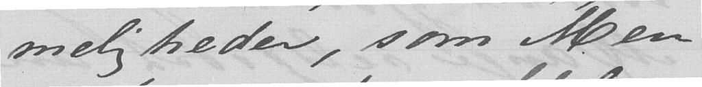meligheder, som Men-
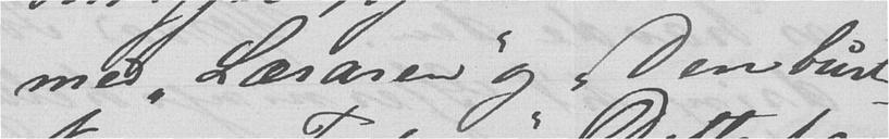med Læraren og Den burt-
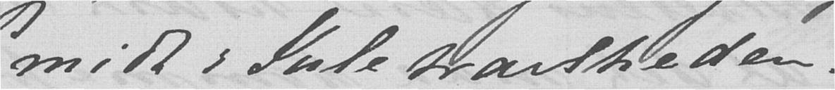midt i Juletravlheden.
